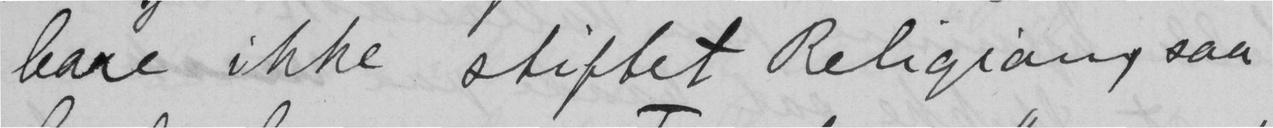bare ikke stiftet Religion, saa
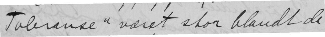Toleranse" været stor blandt de
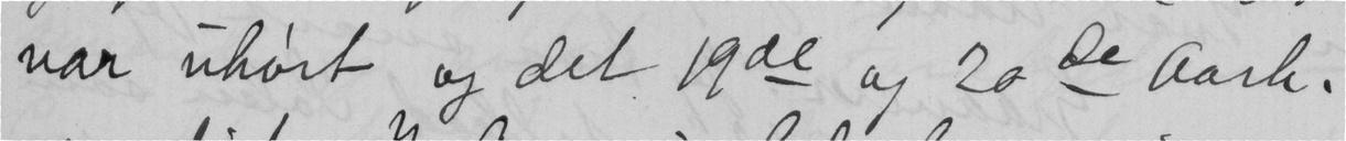var uhørt og det 19de og 20de Aarh.
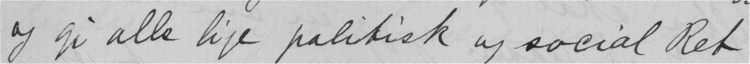og gi alle lige politisk og social Ret
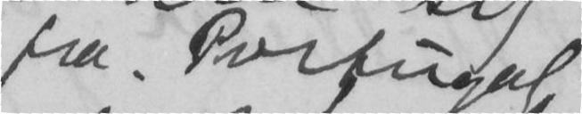fra Portugal
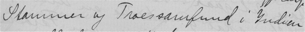Stammer og Troessamfund i Indien
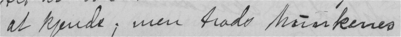at kjende; men trods Munkenes
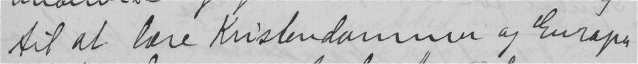til at lære Kristendommen og Europa
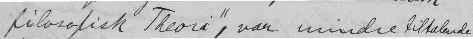filosofisk Theori", var mindre tiltalende
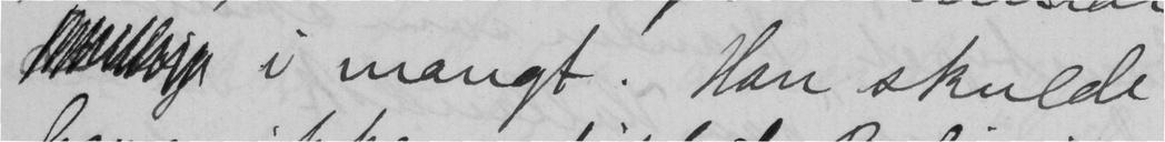i mangt. Han skulde
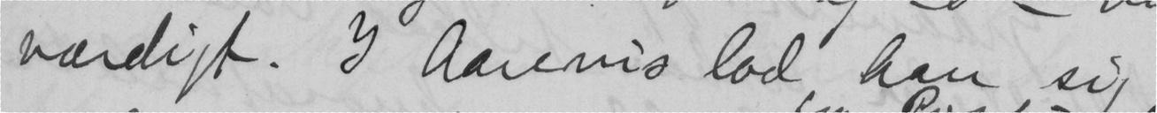værdigt. I Aarevis lod han sig
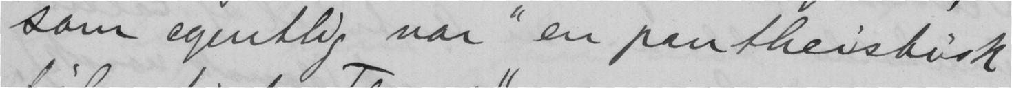som egentlig var "en pantheistisk
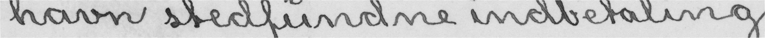havn stedfundne indbetaling
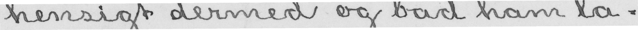hensigt dermed og bad ham la-
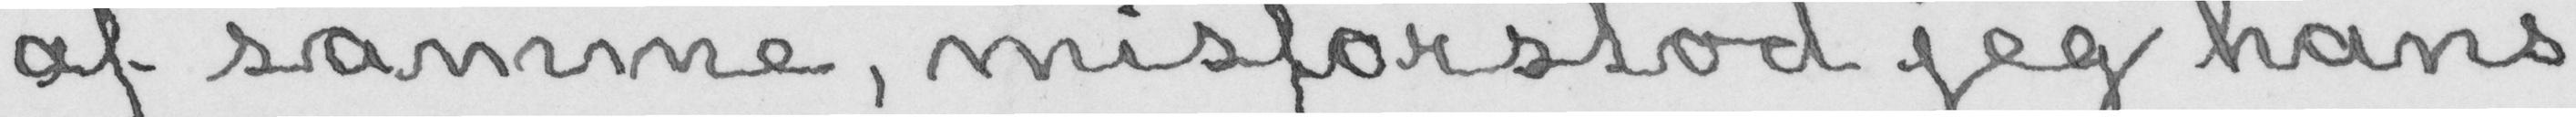af samme, misforstod jeg hans
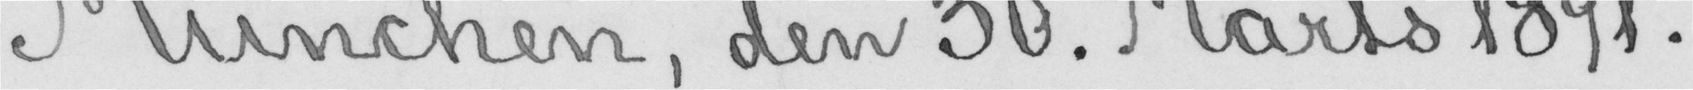München, den 30. Marts 1891.
